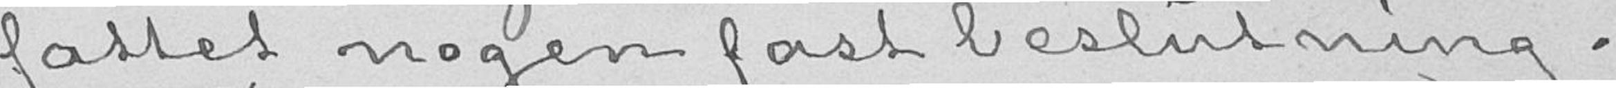fattet nogen fast beslutning.
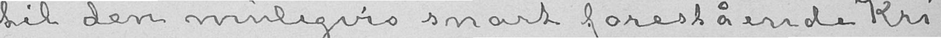til den muligvis snart forestående Kri-
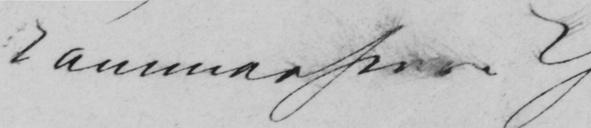Kammerherre
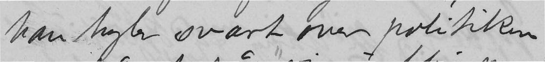han hyler svært over politiken.
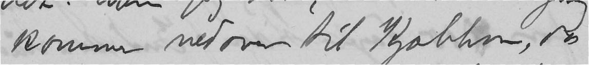kommer nedover til Kjøbhvn, da
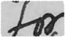For
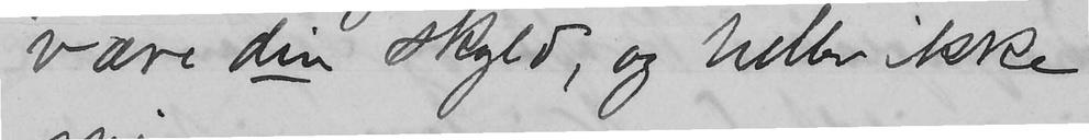være din skyld; og heller ikke
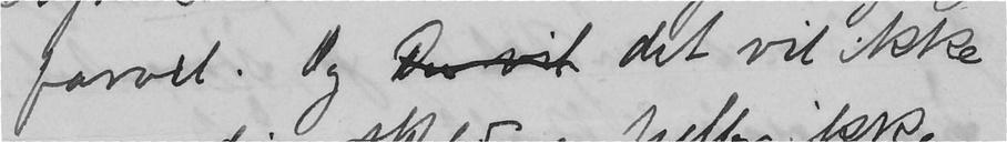farvel. Og der vil det vil ikke
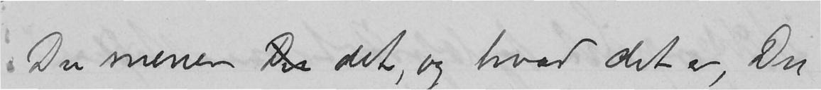Du mener Du det, og hvad det er, Du
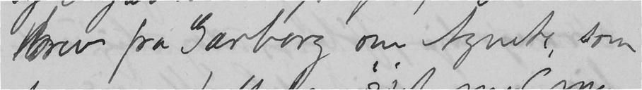brev fra Garborg om Agnete, som
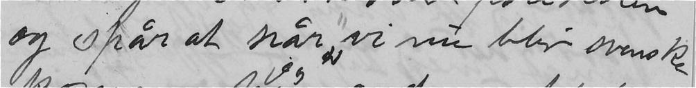og spår at når "vi nu blir svenske
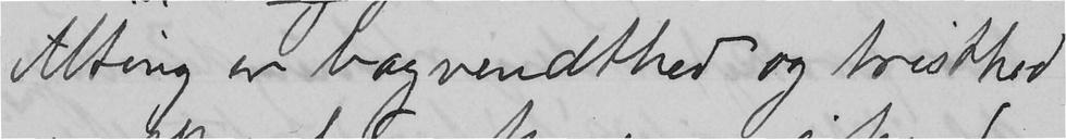Alting er bagvendthed og tristhed
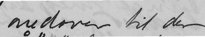nedover til der
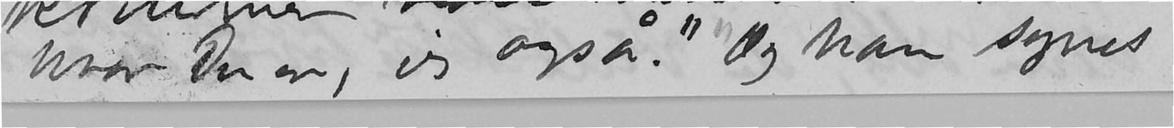hvor Du er, jeg også". Og han synes
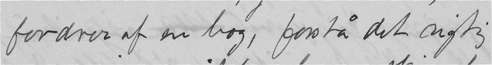fordrer af en bog, forstå det rigtig
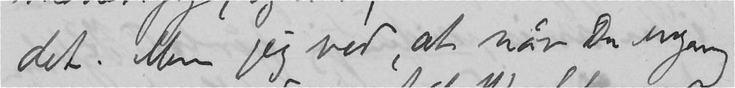det. Men jeg véd, at når du engang
